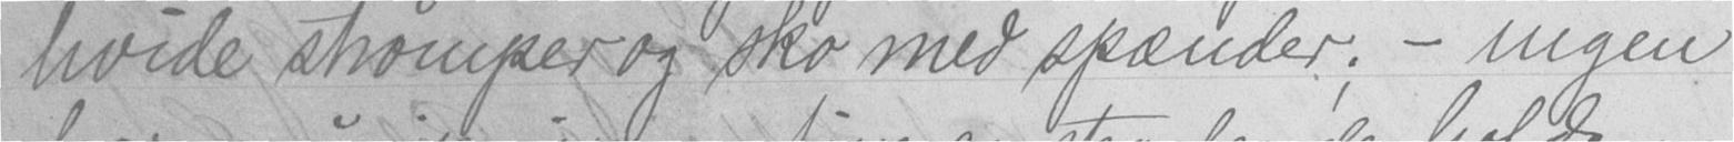hvide strømper og sko med spænder; - ingen
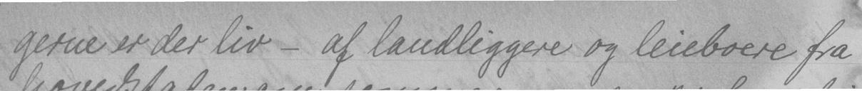gerne er der liv - af landliggere og leieboere fra
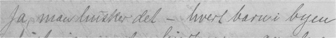Ja, man husker det - hvert barn i byen
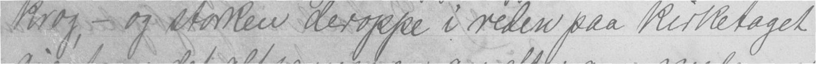krog, - og storken deroppe i reden paa kirketaget
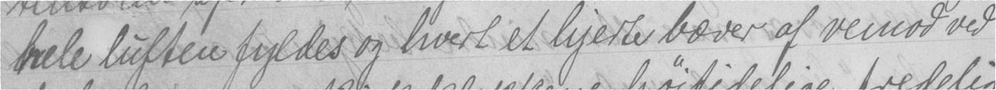hele luften fyldes og hvert et hjerte bæver af vemod ved
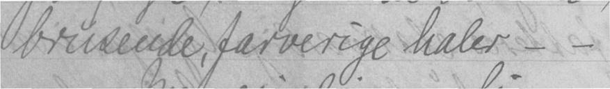brusende, farverige haler - -
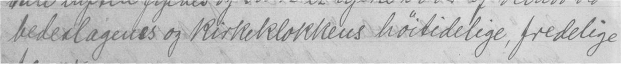bedeslagenes og kirkeklokkens høitidelige, fredelige
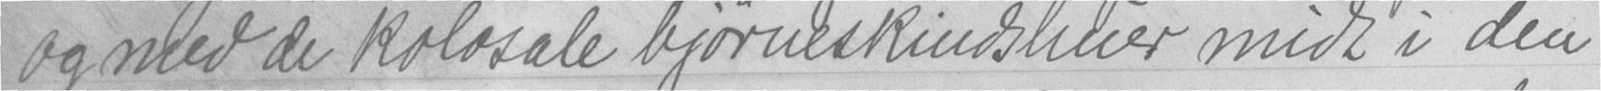og med de kolosale bjørneskindshuer midt i den
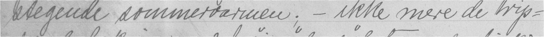stegende sommervarmen; - ikke mere de trip-
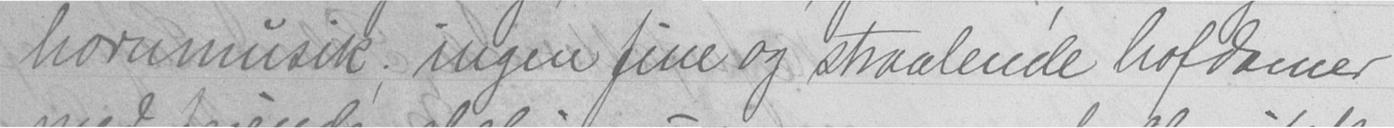hornmusik; ingen fine og straalende hofdamer
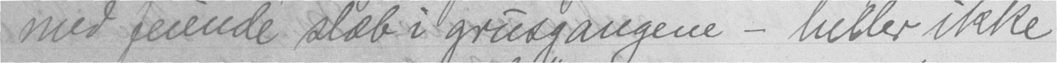med feiende slæb i grusgangene - heller ikke
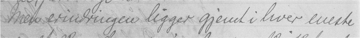Men erindringen ligger gjemt i hver eneste
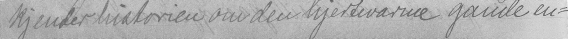kjender historien om den hjertevarme gamle en-
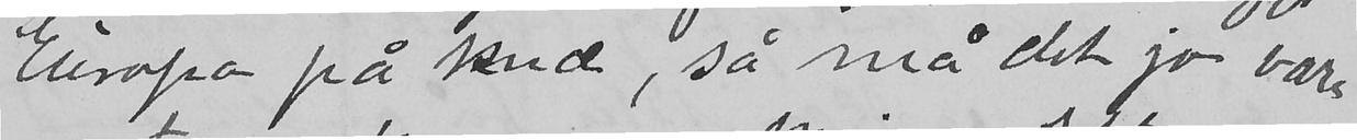Europa på knæ, så må det jo være
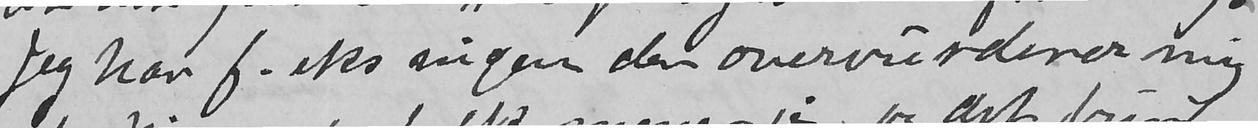Jeg har f. eks ingen den overvurderer mig
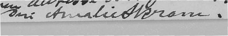din Amalie Skram.
